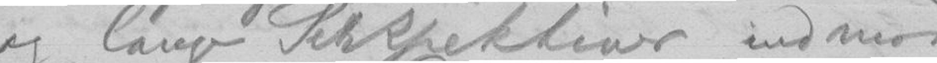og lange Perspektiver ind mod
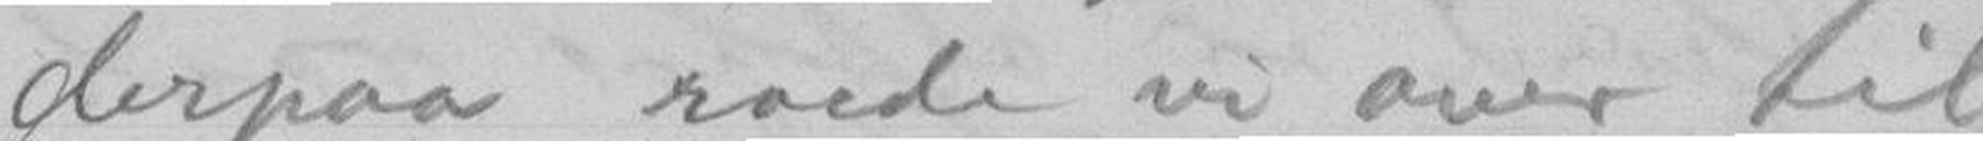derpaa roede vi over til
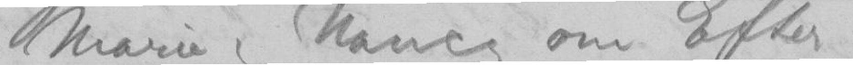Marie og Nancy om Efter-
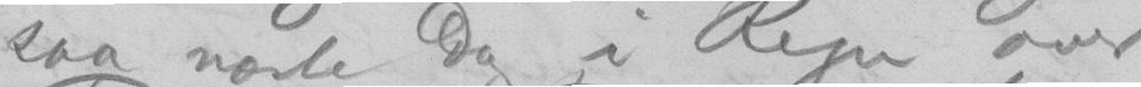saa næste Dag i Regn over
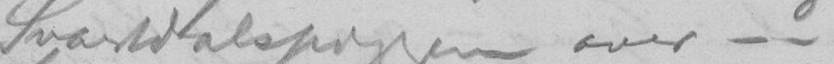Svartdalspiggen over - - -
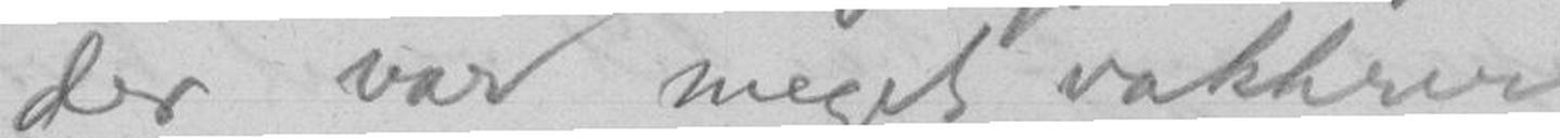der var meget vakkrere,
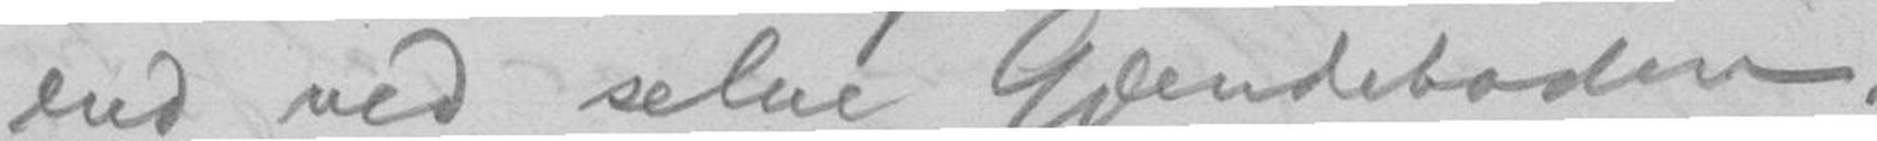end ved selve Gjendeboden.
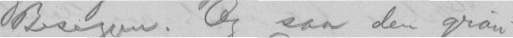Beseggen. Og saa den grøn-
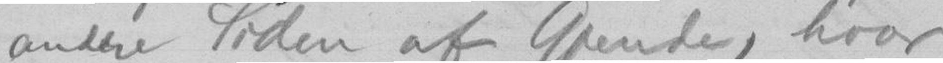andre Siden af Gjende, hvor
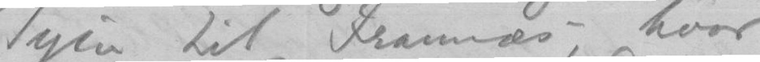Tyin til Framnæs, hvor
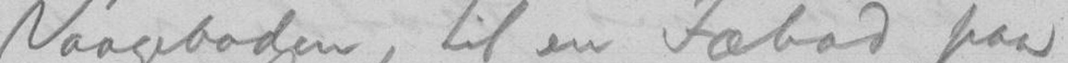Vaageboden, til en Fæbod paa
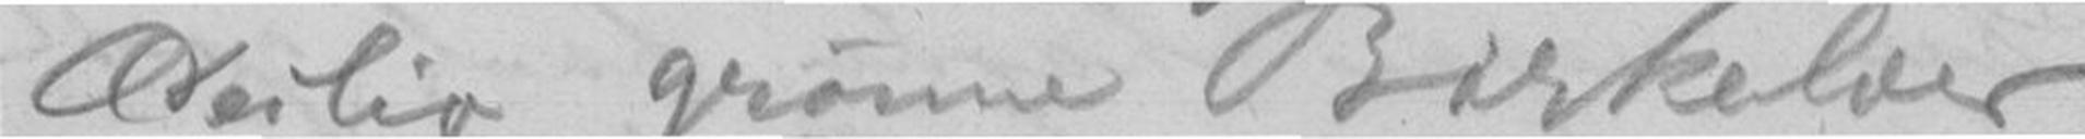Deilige grønne Birkelier
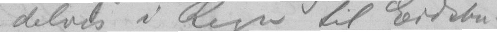delvis i Regn til Eidsbu-
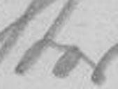stor,
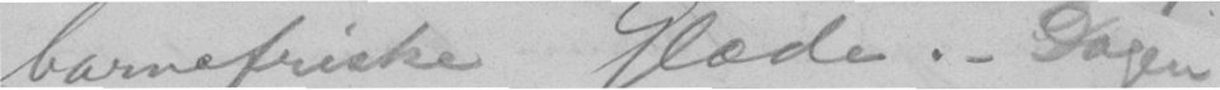barnefriske Glæde. - Dagen
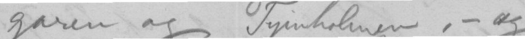garen og Tyinholmen, - og
